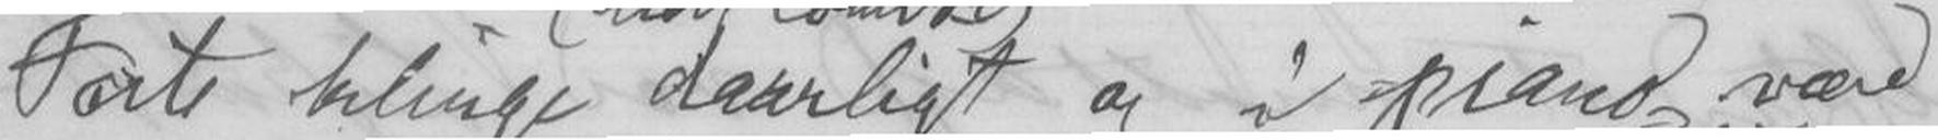Forte klinge daarligt og i piano være
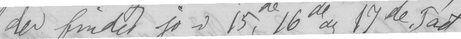der findes jo i 15de, 16de og 17de Tact
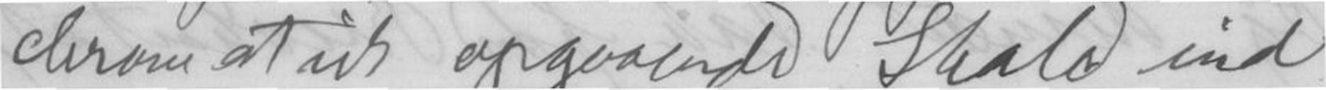chromatisk opgaaende Skala ind
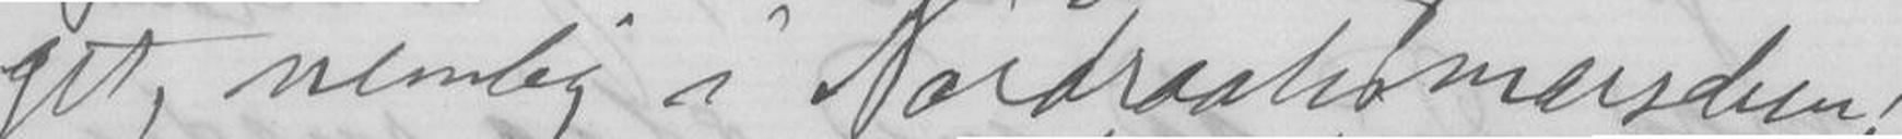get, nemlig i Nodraaksmarsdsen;
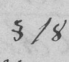§ 18
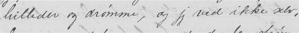billeder og drømme, og jeg ved ikke selv,
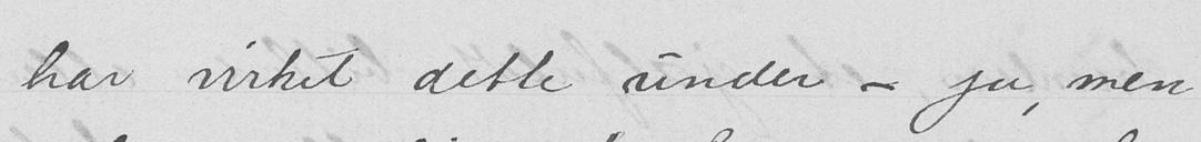har virket dette under - ja, men
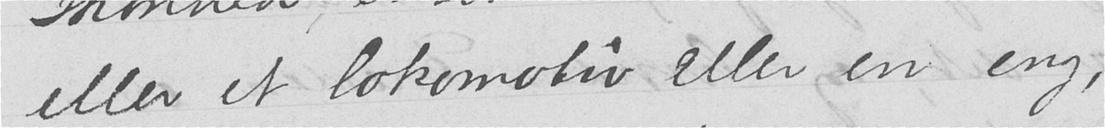eller et lokomotiv eller en eng,
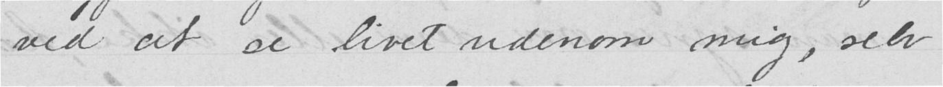ved at se livet udenom mig, selv
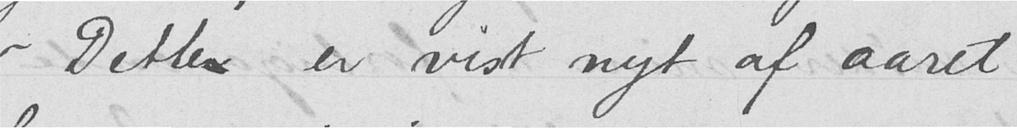- Dette er vist nyt af aaret
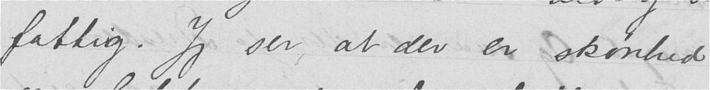fattig. Jeg ser, at der er skønhed
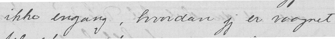ikke engang, hvordan jeg er vaagnet
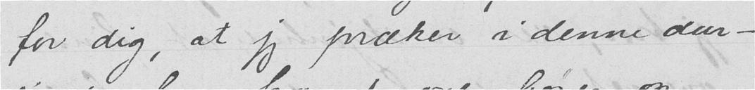for dig, at jeg præker i denne dur -
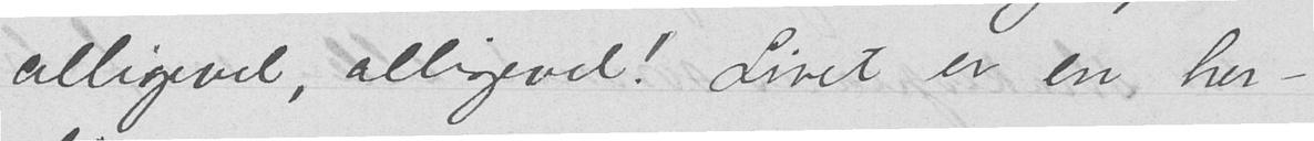alligevel, alligevel! Livet er en her-
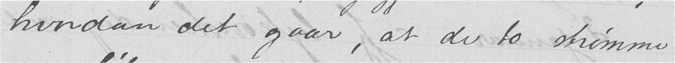hvordan det gaar, at de to strømmer
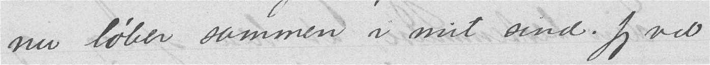nu løber sammen i mit sind. Jeg ved
